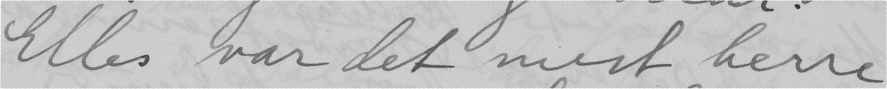Elles var det mest berre
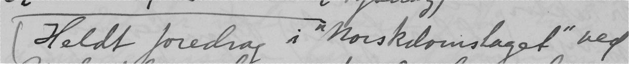Heldt foredrag i "Norskdomslaget" ved
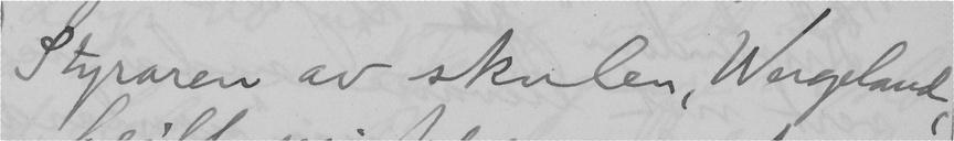Styraren av skulen, Wergeland,
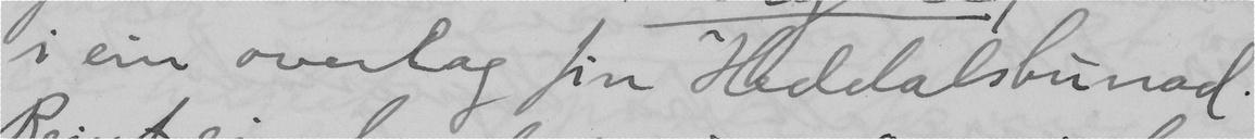i ein overlag fin Heddalsbunad.
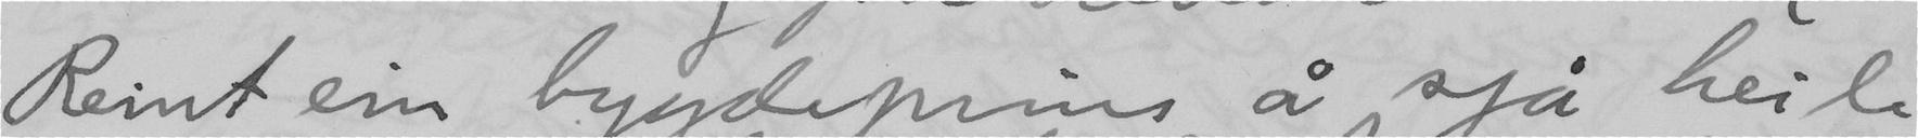Reint ein bygdeprins å sjå heile
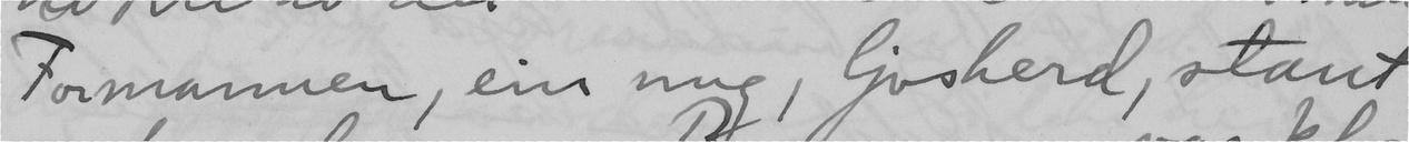Formannen, ein ung, ljosherd, staut
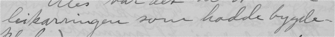leikarringen som hadde bygde-
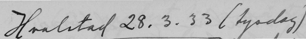Hvalstad 28.3.33 (tysdag)
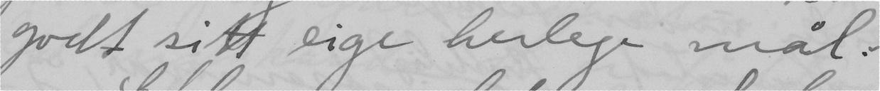godt sitt eige herlege mål.
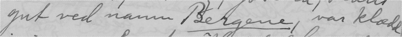gut ved namn Bergene, var klæde
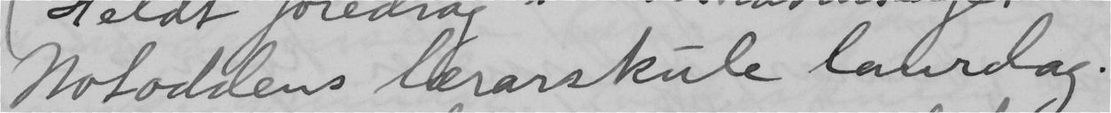Notoddens lerarskule laurdag.
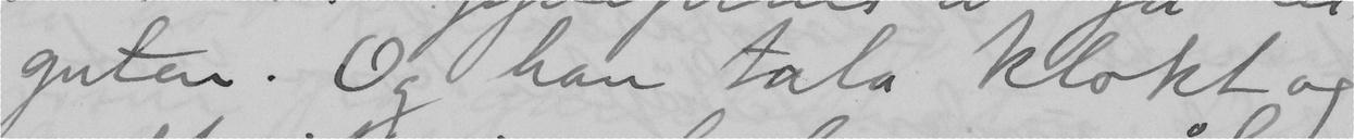guten. Og han tala klokt og
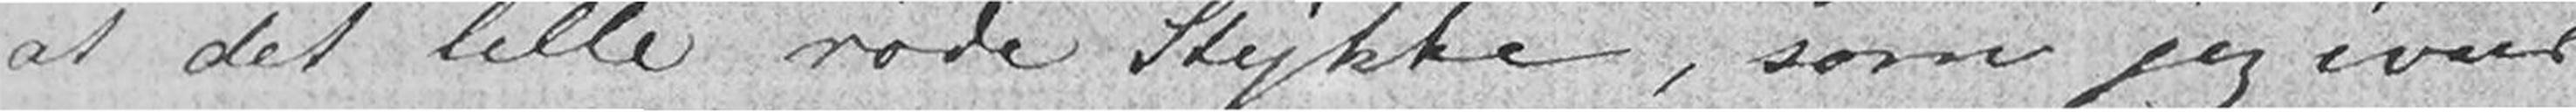at det lille røde Stykke, som jeg ivaar
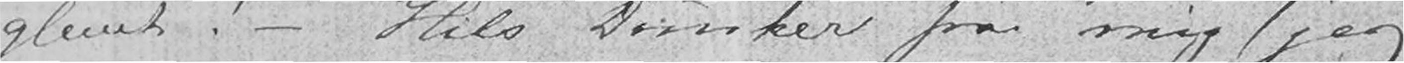glemt!- Hils Dunker fra mig (jeg
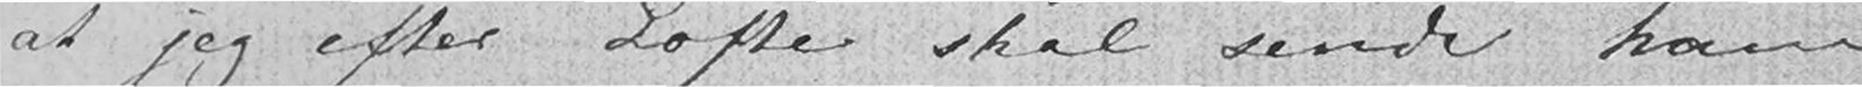at jeg efter Løfte skal sende ham
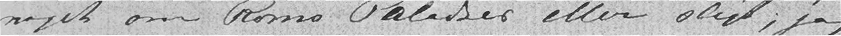noget om Roms Paladser eller sligt; jeg
